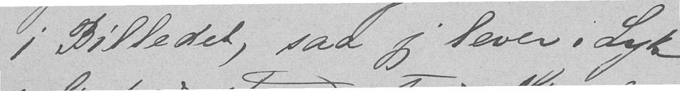i Billedet, saa jeg lever i Lyk-
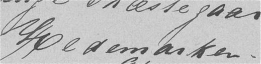Hedemarken.
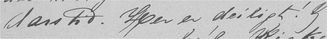Aarstid. Her er deiligt! Jeg
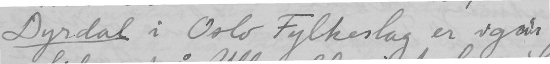Dyrdal i Oslo Fylkeslag er igår
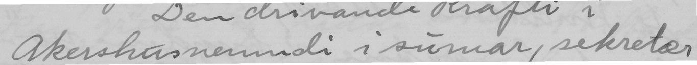Akershusnemndi i sumar, sekretær
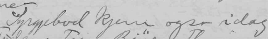Syrgjebod kjem ogso idag
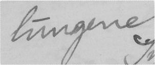lungene.
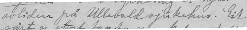avliden på Ullevald sjukehus. Eit
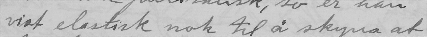vist elastisk nok til å skyna at
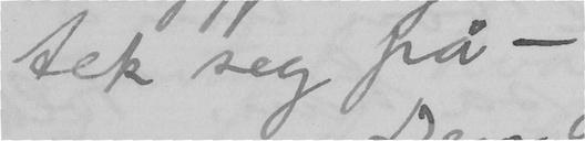tek seg på.
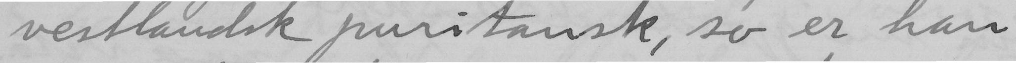vestlandsk puritansk, so er han
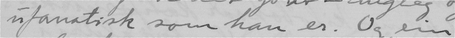ufanatisk som han er. Og ein
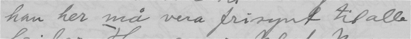han her må vera frisynt til alle
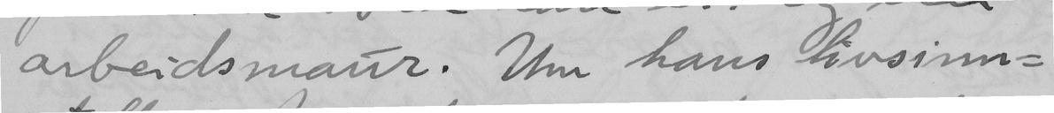arbeidsmaur. Um hans livsinn-
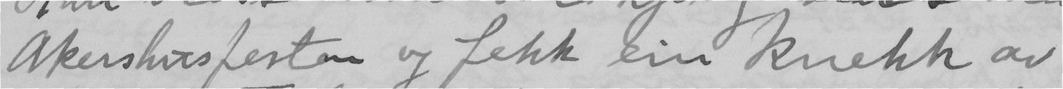Akershusfesten og fekk ein knekk av
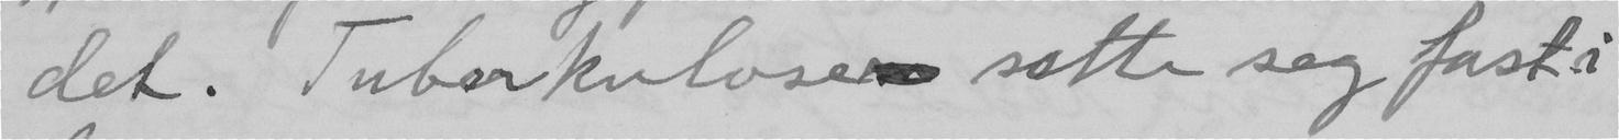det. Tuberkulosen sette seg fast i
